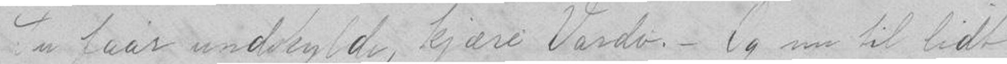Du faar undskylde, kjære Vardo.- Og no til lidt
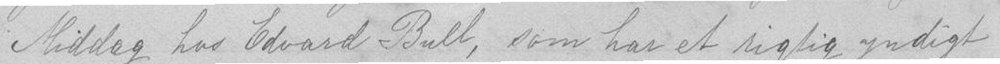Middag hos Edvard Bull, som har et rigtig yndigt
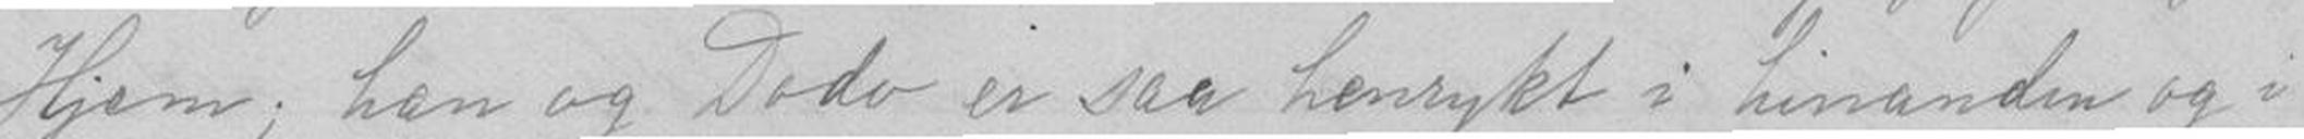Hjem; han og. Dodo er saa henrykt i hinanden og i
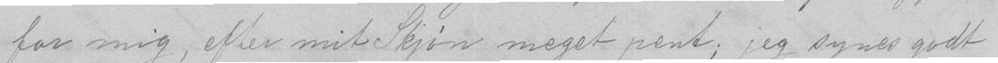for mig, efter mit skjøn meget pent; jeg synes godt
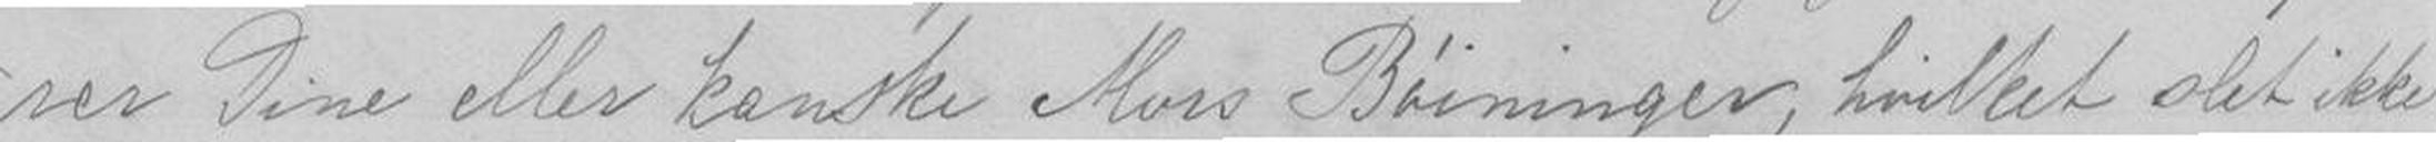rer Dine eller kanske Mors Bøininger, hvilket det ikke
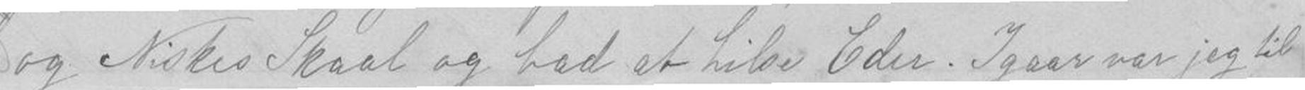og Niskes Skaal og bad at hilse Eder. Igaar var jeg til
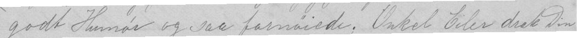godt Humør og saa fornøiede; Onkel Eiler drak Din
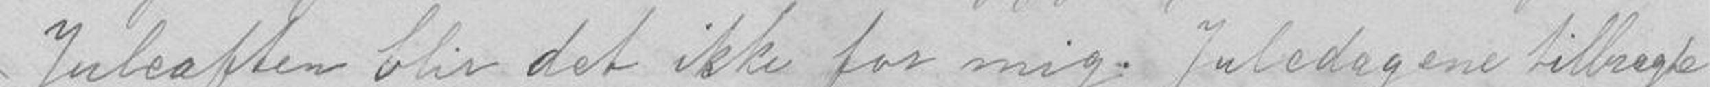Juleaften blir det ikke for mig. Juledagene tilbragte
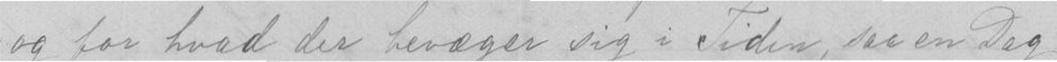og for hvad der bevæger sig i Tiden, saa en Dag
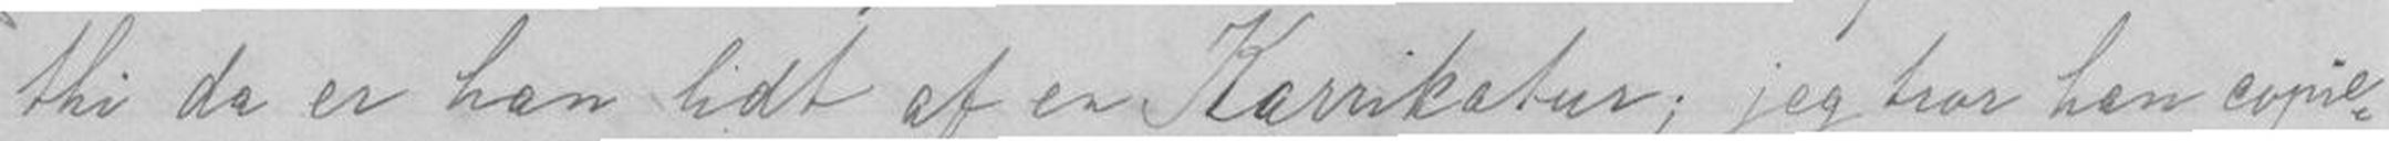thi da er han lidt af en Karikatur; jeg tror han copie-
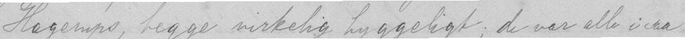Hagempo, begge virkeligt hyggeligt; de var alle i saa
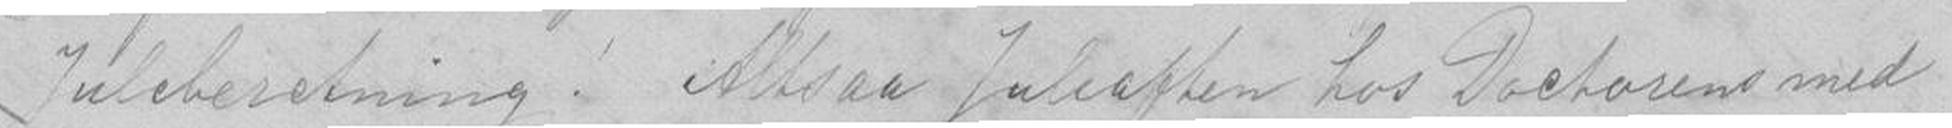Juleberetning! Altsaa Juleaften hos Doctorens med
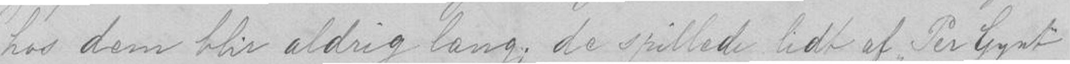hos dem blir aldrig lang; de spillede lidt af "Per Gynt"
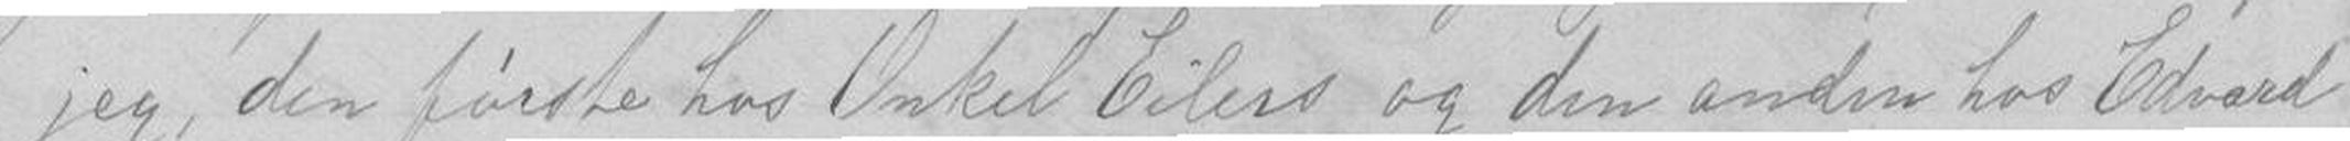jeg, den første hos Onkel Eilers og den andre hos Edvart
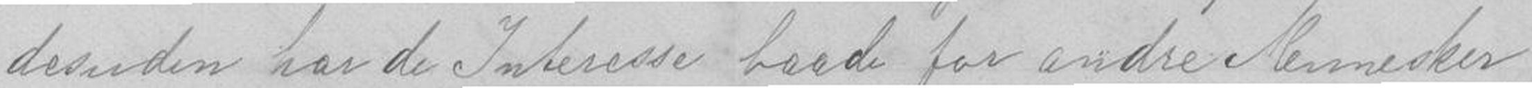desuden har de Interesse baade for andre Mennesker
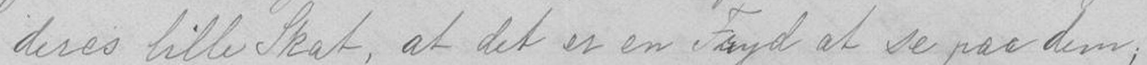deres lille Skat, at det er en Fryd at se paa dem;
